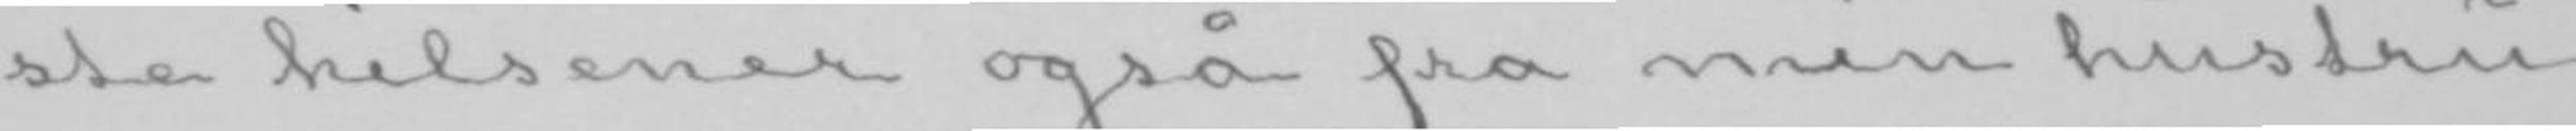ste hilsener også fra min hustru
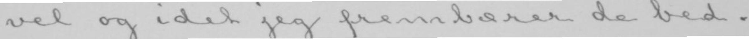vel og idet jeg frembærer de bed-
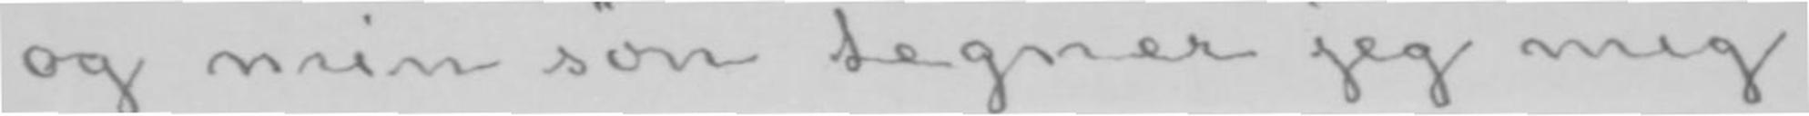og min søn tegner jeg mig
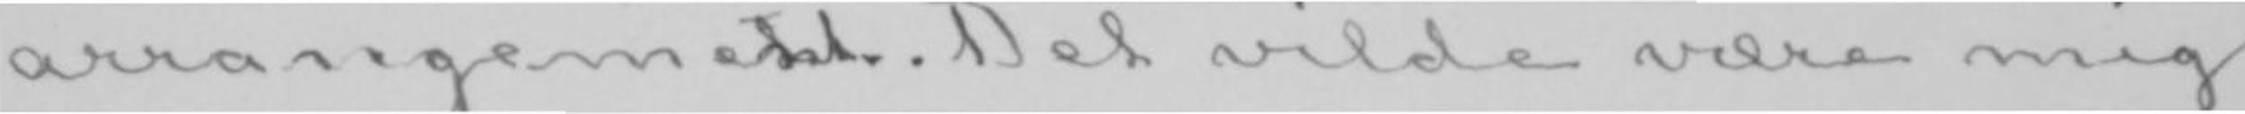arrangemetnt. Det vilde være mig
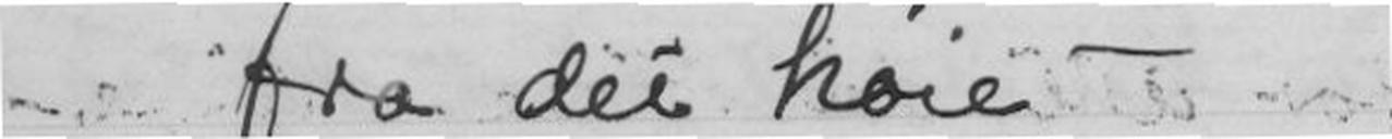fra det høie -
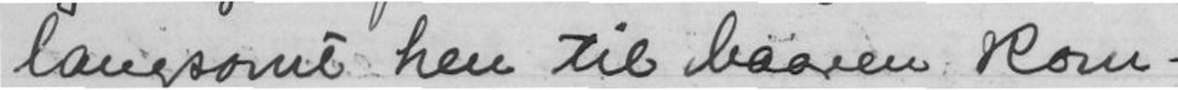langsomt hen til baaren kom -
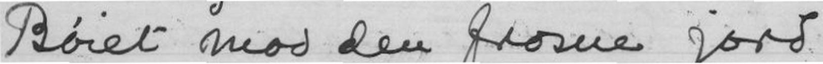Bøiet mod den frosne jord
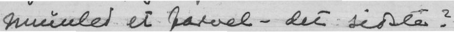mumlet et farvel - det sidste?
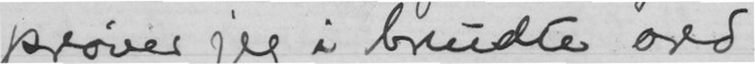prøver jeg i brudte ord
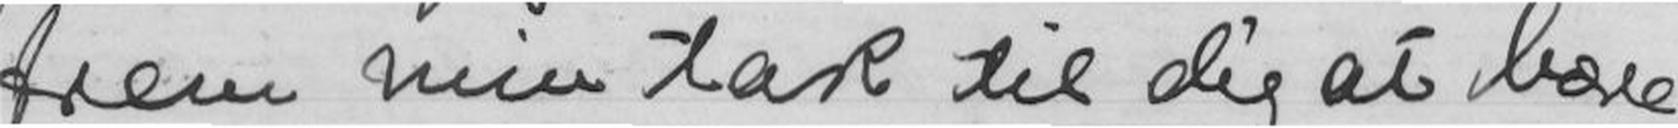frem min tak til dig at bære
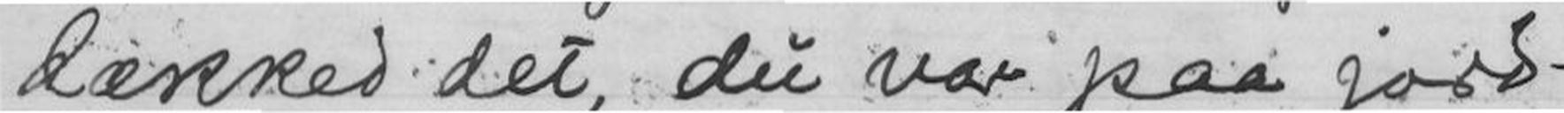dækked det, du var paa jord -
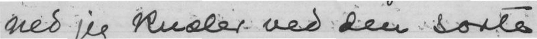ned jeg rusler ved den sorte
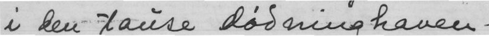i den tause dødninghaven -
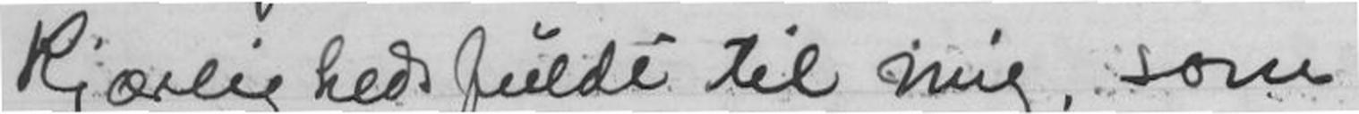Kjærlighedsfuldt til mig, som
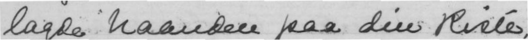lagde haanden paa din kiste,
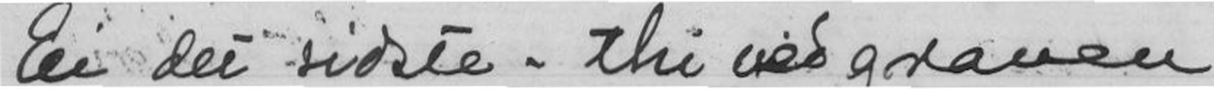Ei det sidste - thi ved graven
